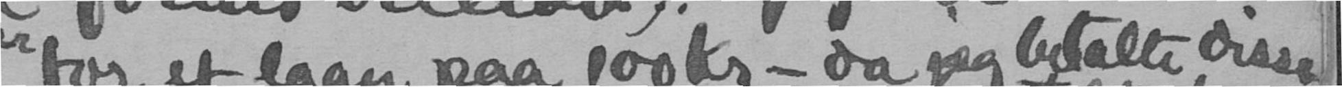for et laan paa 100Kr - da paa betalte disse)
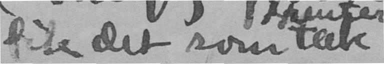fik det som tak
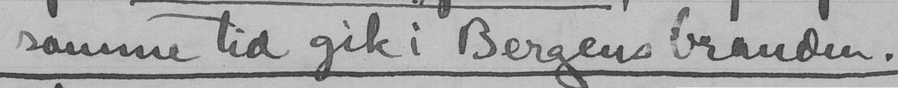samme tid gik i Bergens branden.
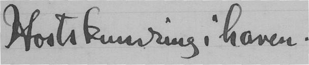"Høstskumring i haven."
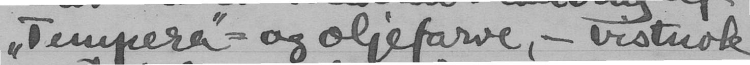"tempera"- og oljefarve, - vistnok
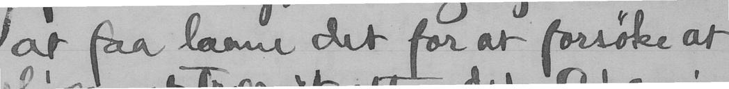at faa laane det for at forsøke at
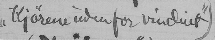("Kjørene udenfor vinduet")
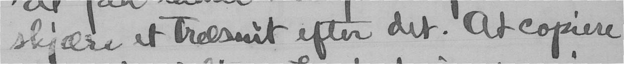skjære et træsnit efter det. At copiere
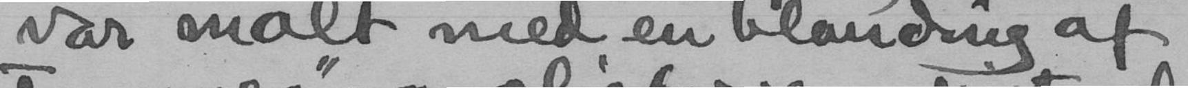var malt med en blanding af
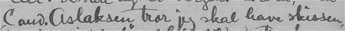Cand. Aslaksen tror jeg skal have skissen,
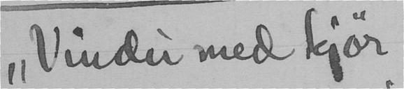"Vindu med kjør"
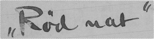"Rød nat"
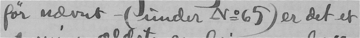for nævnt - (under No 65) er det et
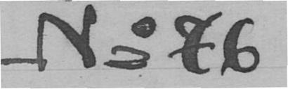No 76
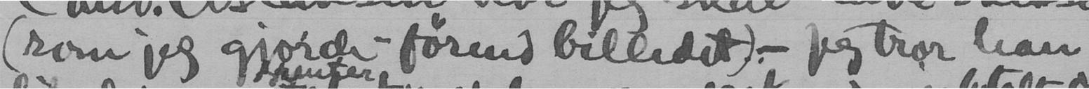(som jeg gjorde - førend billedet) - jeg tror han
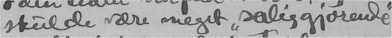skulde være meget "saliggjørende"
